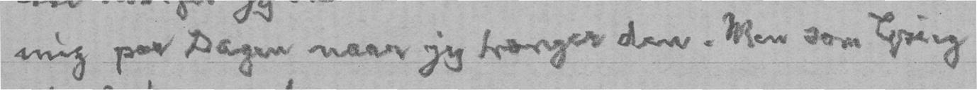mig paa Dagen naar jeg trænger den. Men som Grieg
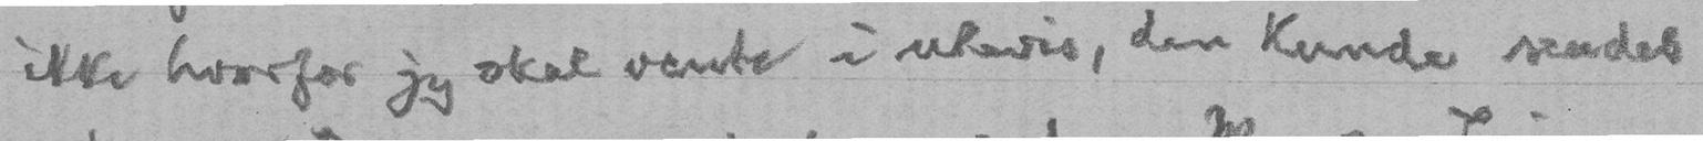ikke hvorfor jeg skal vente i ukevis, den kunde sendes
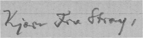Kjære Fru Stray,
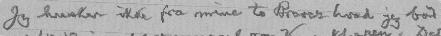Jeg husker ikke fra mine to Brever hvad jeg bad
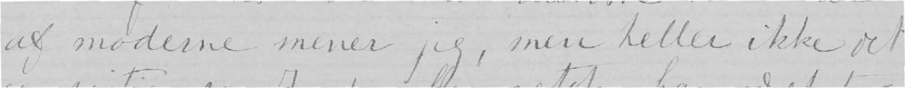af moderne mener jeg, men heller ikke det
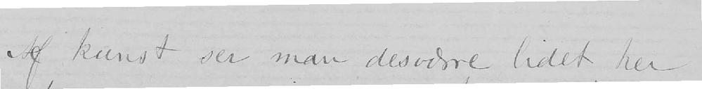Af kunst ser man desværre lidet her
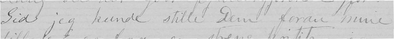Gid jeg kunde stille Dem foran mine
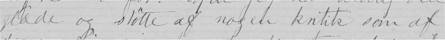glæde og støtte af nogen kritik som af
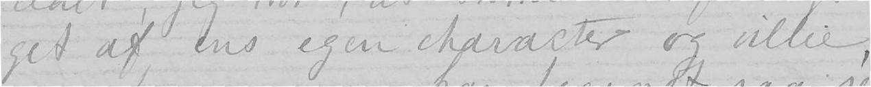get af ens egen character og villie,
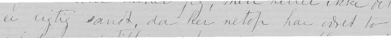er rigtig sandt, da her netop har været to
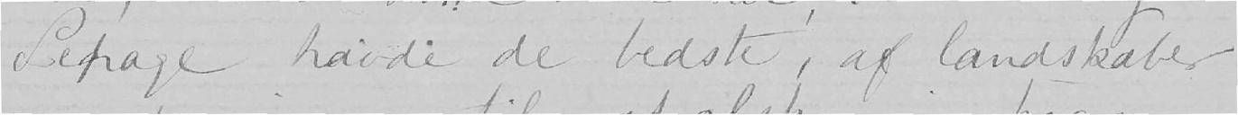Lepage havde de bedste, af landskaber
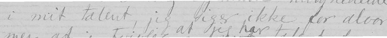i mit talent, jeg siger ikke for alvor
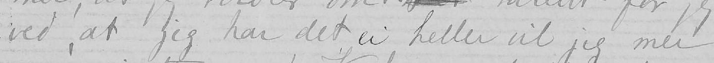ved, at jeg har det, ei heller vil jeg mer
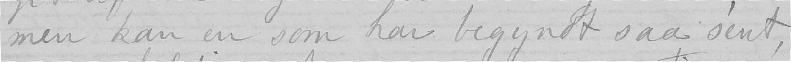men kan en som har begyndt saa sent,
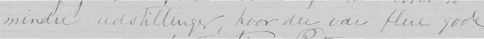mindre udstillinger, hvor der var flere gode
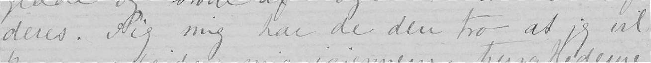deres. Sig mig har de den tro at jeg vil
